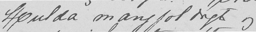Hulda mangfoldigt og
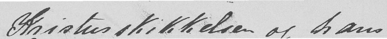Kristusskikkelsen og hans
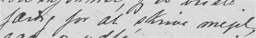færdig for at skrive meget,
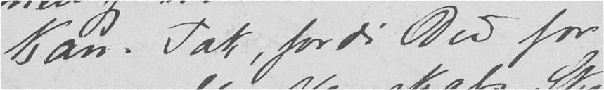kan. Tak, fordi Du for
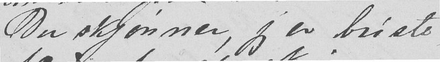Du skjønner, jeg er briste-
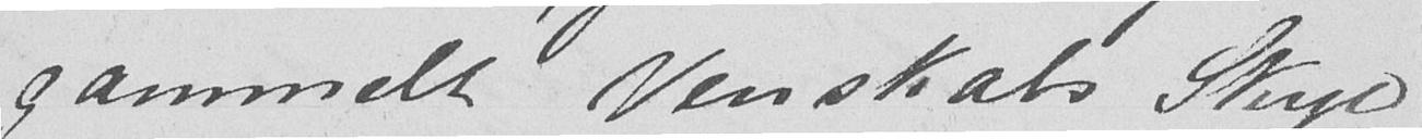gammelt Venskabs Skyld
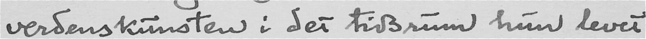verdenskunsten i det tidsrum hun levet
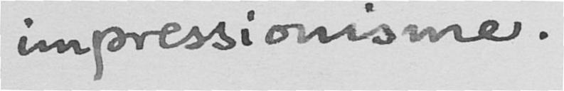impressionisme.
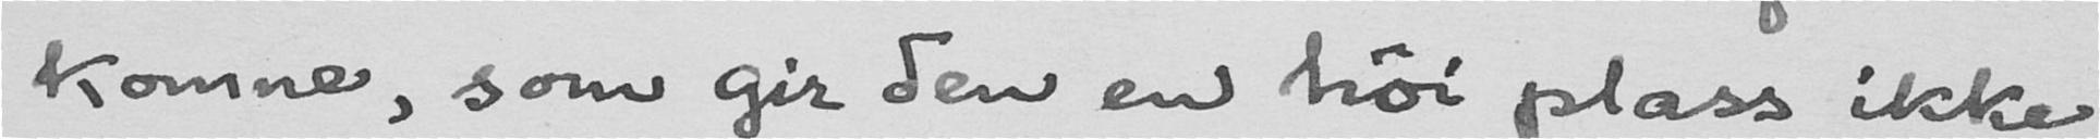komne, som gir den en høi plass ikke
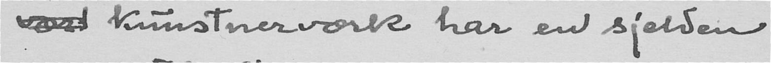værd kunstnerværk har en sjelden
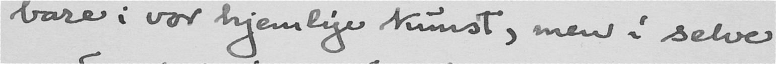bare i vor hjemlige kunst, men i selve
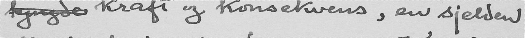tyngde kraft og konsekvens, en sjelden
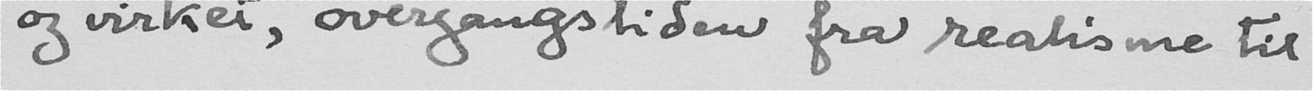og virket, overgangstiden fra realisme til
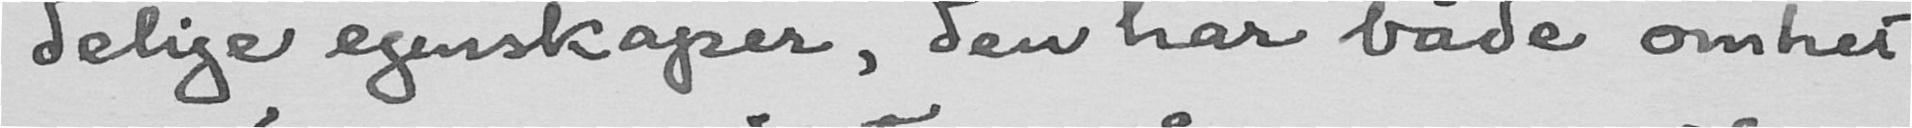delige egenskaper, den har både ømhet
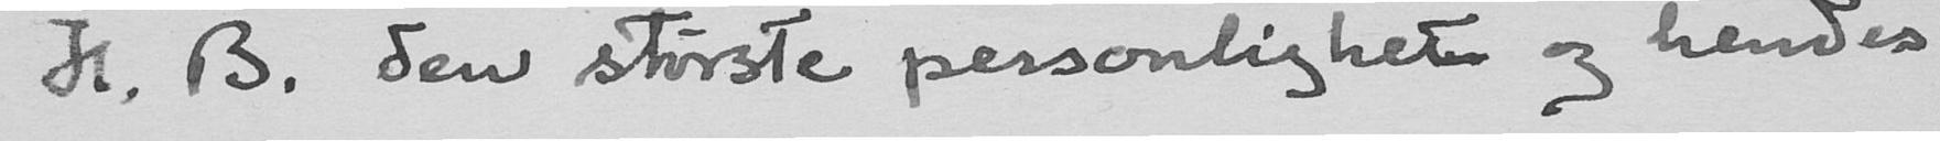H. B. den største personlighet og hendes
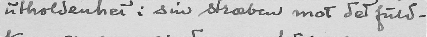utholdenhet i sin stræben mot det fuld-
